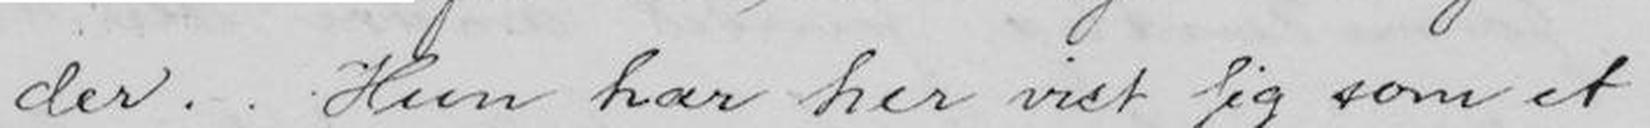der. Hun har her vist sig som et
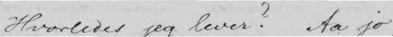Hvorledes jeg lever? Aa jo,
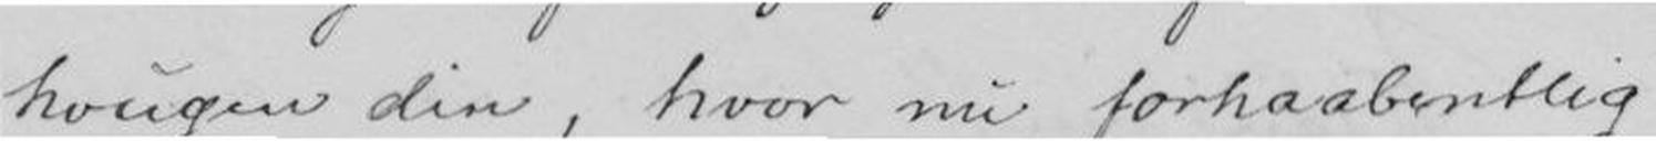haugen din, hvor nu forhaabentlig
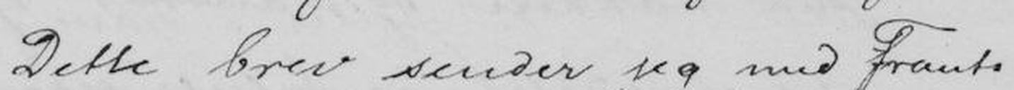Dette brev sender jeg med Frants
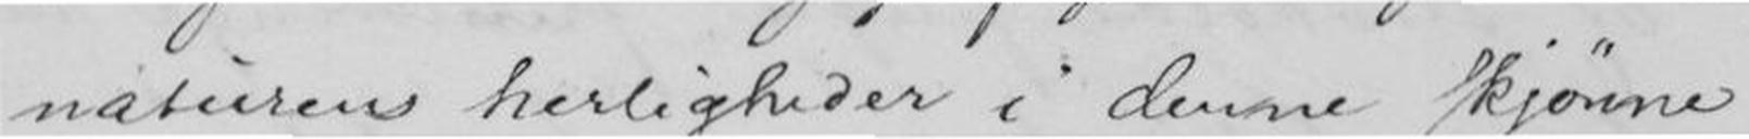naturens herligheder i denne skjønne
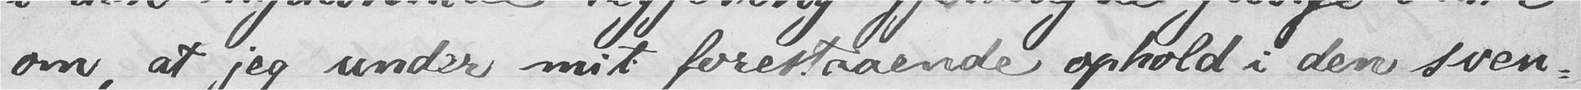om, at jeg under mit forestaaende ophold i den sven-
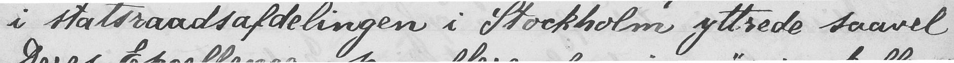i statsraadsafdelingen i Stockholm yttrede saavel
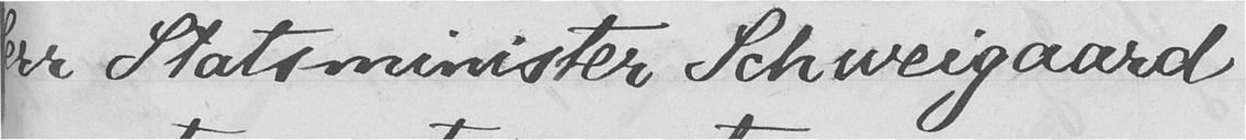Herr Statsminister Schweigaard
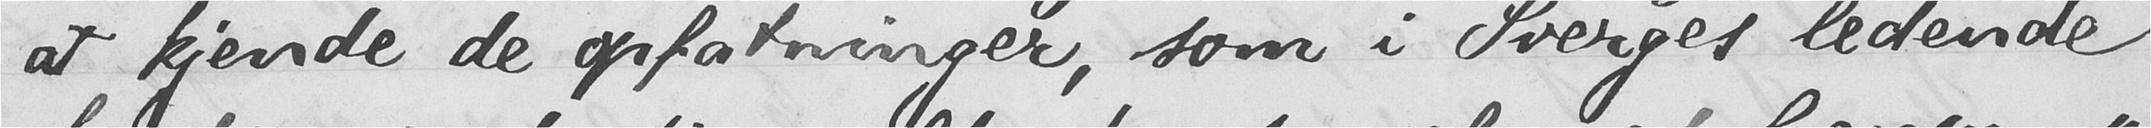at kjende de opfatninger, som i Sverges ledende
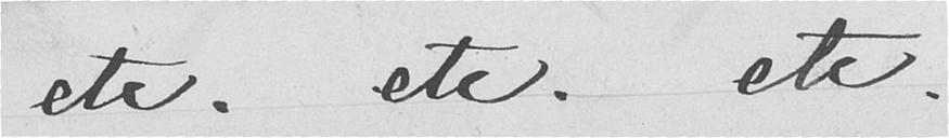etc. etc. etc.
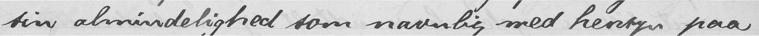sin almindelighed som navnlig med hensyn paa
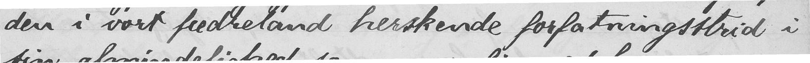den i vort fedreland herskende forfatningsstrid i
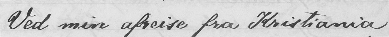Ved min afreise fra Kristiania
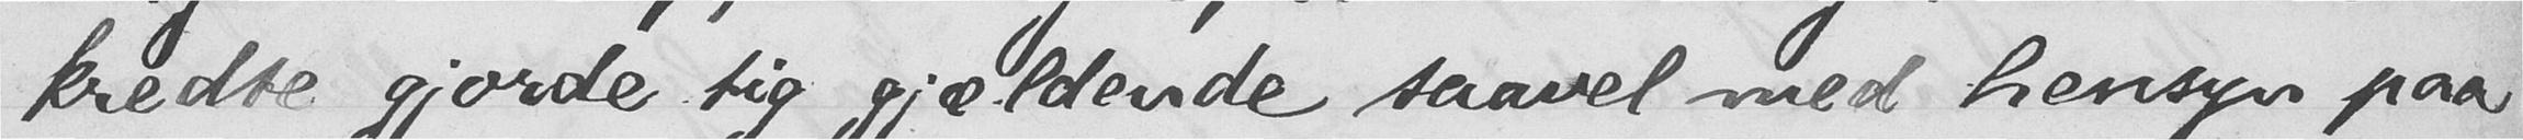kredse gjorde sig gjældende saavel med hensyn paa
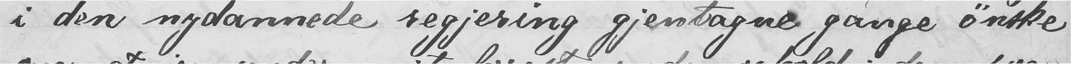i den nydannede regjering gjentagne gange ønske
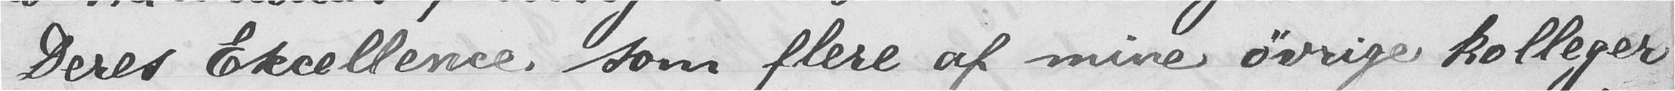Deres Excellence som flere af mine øvrige kolleger
